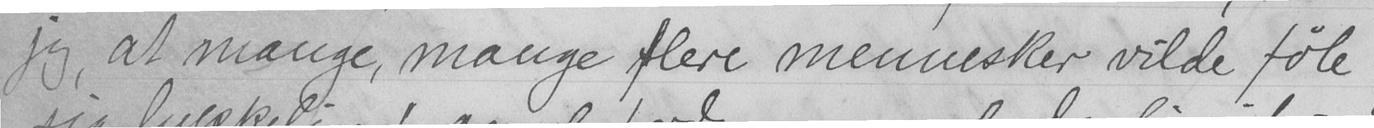jeg, at mange, mange flere mennesker vilde føle
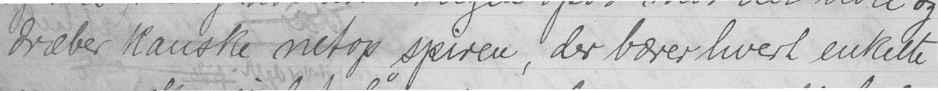dræber kanske netop spiren, der bærer hvert enkelte
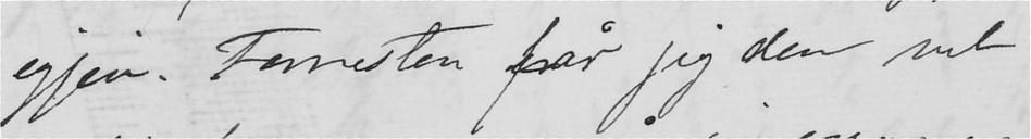igjen! Forresten får jeg den vel
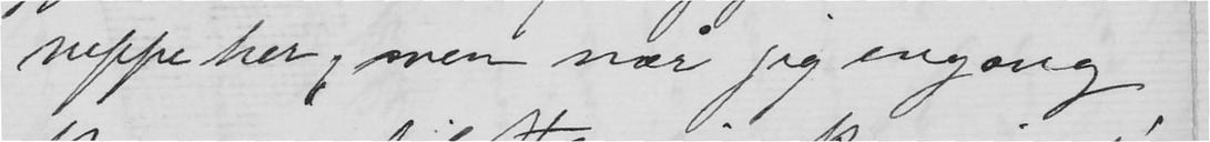neppe her, men når jeg engang
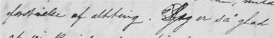forståelse af alting. Jeg er så glad
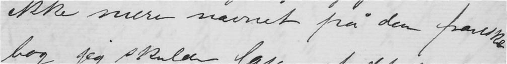ikke mere navnet på den franske
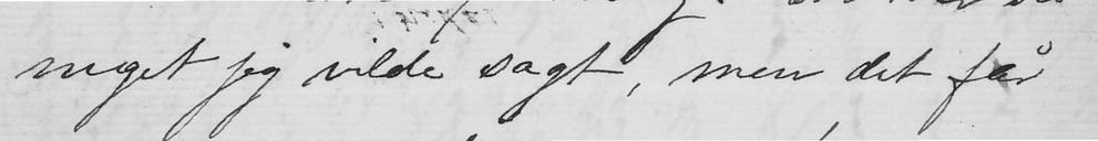meget jeg vilde sagt, men det får
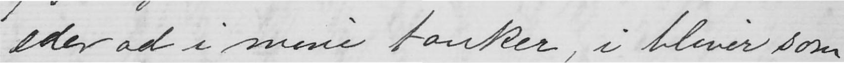eder ad i mine tanker, i bliver som
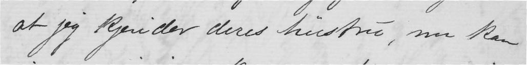at jeg kjender deres hustru, nu kan
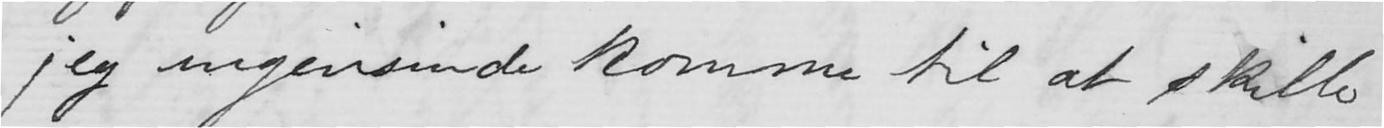jeg ingensinde komme til at skille
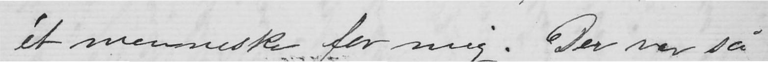ét menneske for mig. Der var så
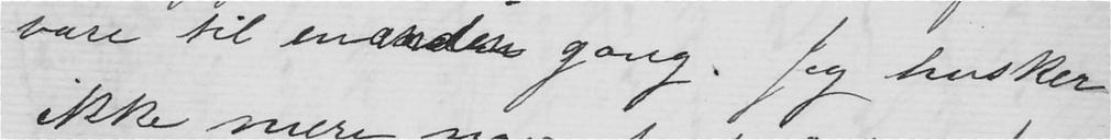være til en anden gang. Jeg husker
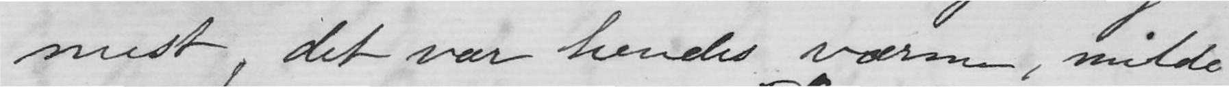mest, det var hendes varme, milde
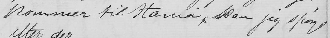kommer til Xania, kan jeg spørge
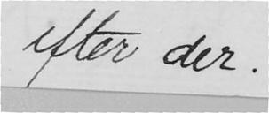efter den.
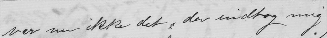var nu ikke det, der indtog mig
